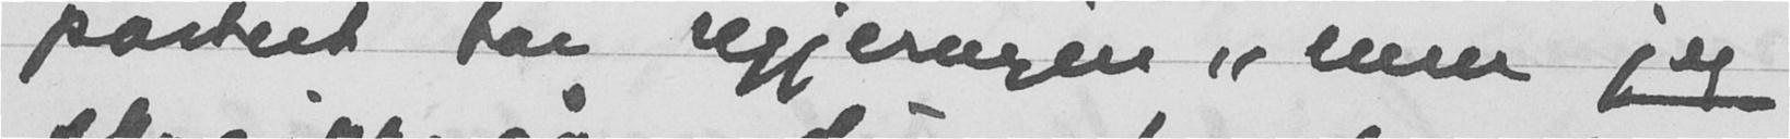partiet tar regjeringen "men jeg
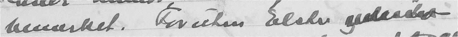bemerket. Frøken Elster
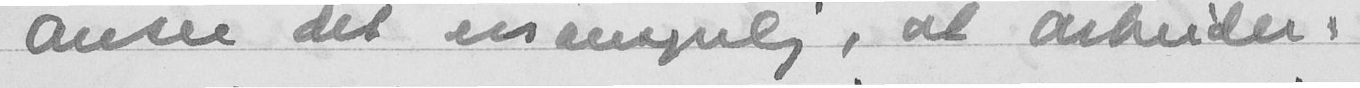anser det en mulighet, at arbeider-
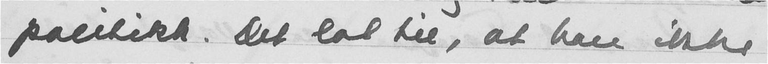politikk. Det lot til, at han ikke
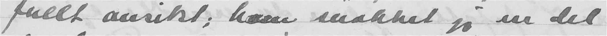fullt ansikt; ham snakket jeg en del
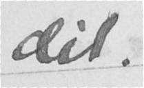dit.
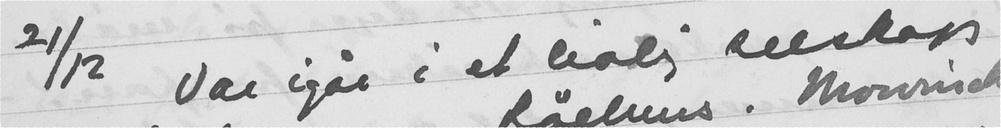21/12 Var igår i et livlig selskap
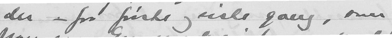der - for første og siste gang, som
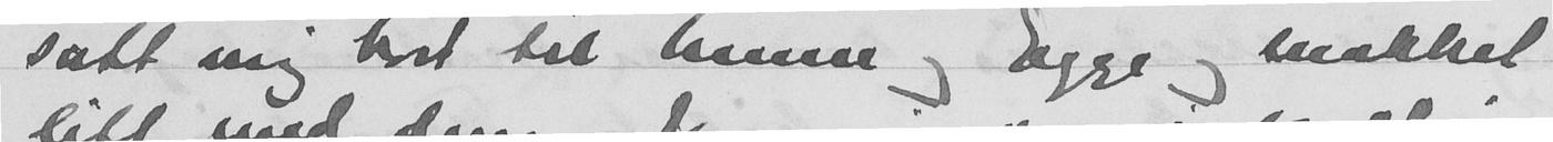satt mig bort til henne og Egge og snakket
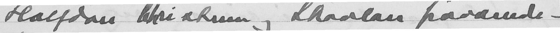Halfdan Christenn og Skavlan fraværende -
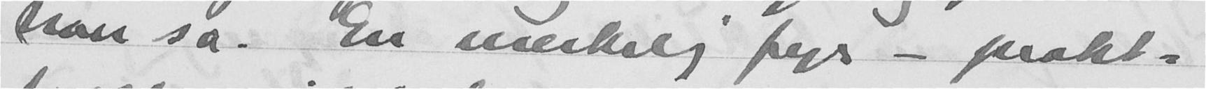han sa. En merkelig fyr - prakt-
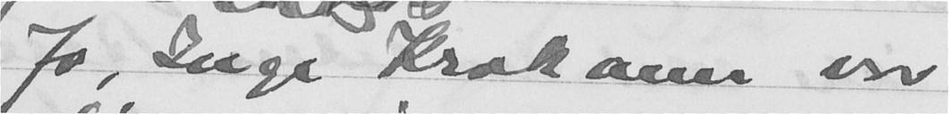Ja, Inge Krokann som var
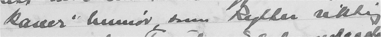kaner" humør som Rytter riktig
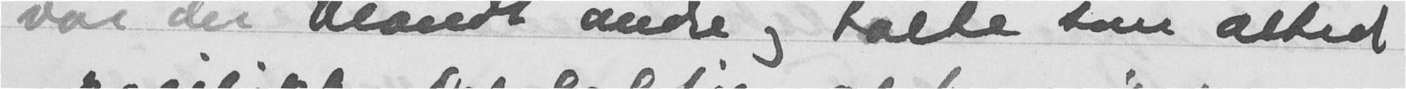var der blandt andre og talte som altid
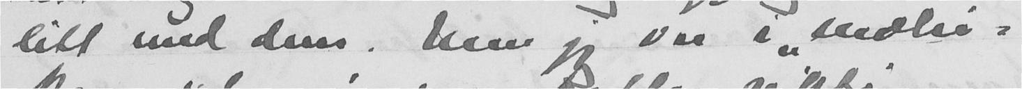litt med dem. Men jeg var i "molie-
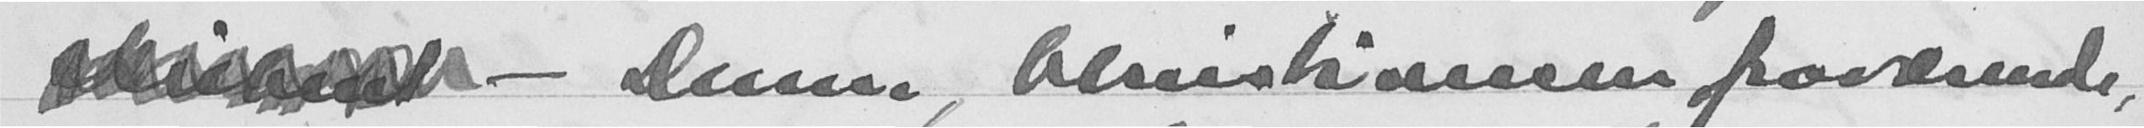- Duun, Kristiansen fraværende,
